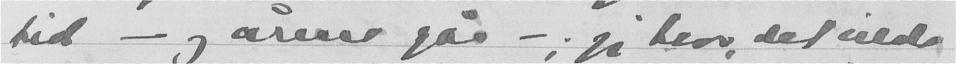tid - og årene går - jeg tror, det vilde
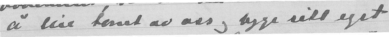å leie tunet av oss og bygge sitt eget
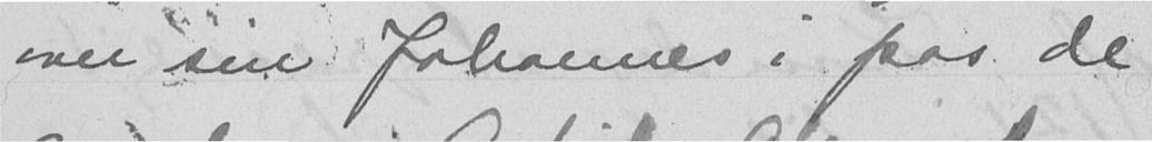over sin Johannes i pas de
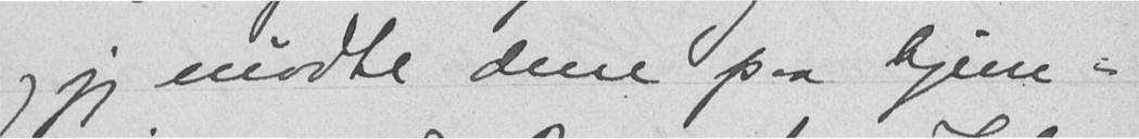og jeg mødte dem paa hjem-
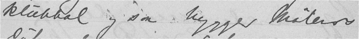klubbal og saa brygger Møllers
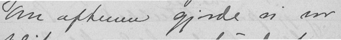Om aftenen gjorde vi vor
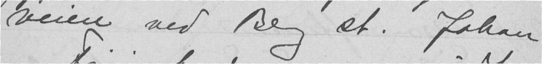veien ved Berg st. Johan
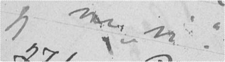jeg var "vi"
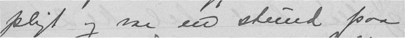pligt og var en stund paa
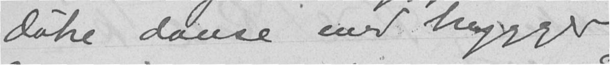døtre danse med brygger
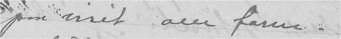paa visit om form.
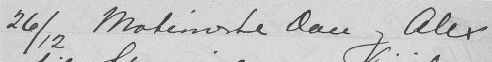26/12 Motionerte Don og Alex
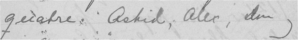quatre. Astrid, Alex, Don og
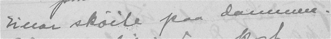Einar skøite paa dammen.
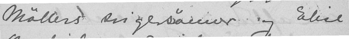Møllers svigersønner og Elise
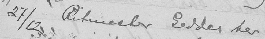27/12 Ritmester Geddes her
